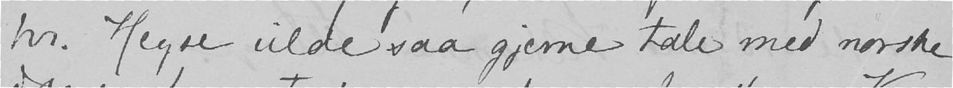hr. Heyse vilde saa gjerne tale med norske
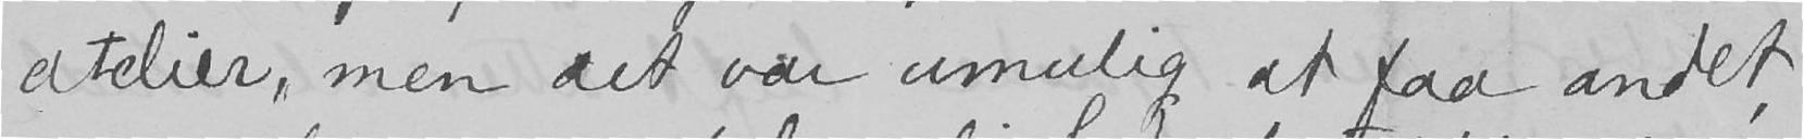atelier, men det var umulig af faa andet,
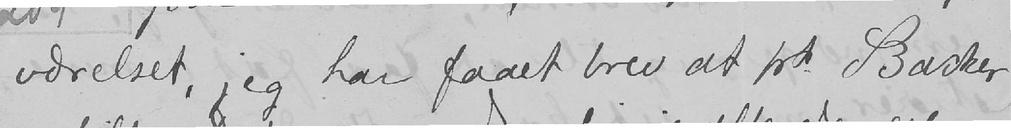værelset, jeg har faaet brev at frk. Backer
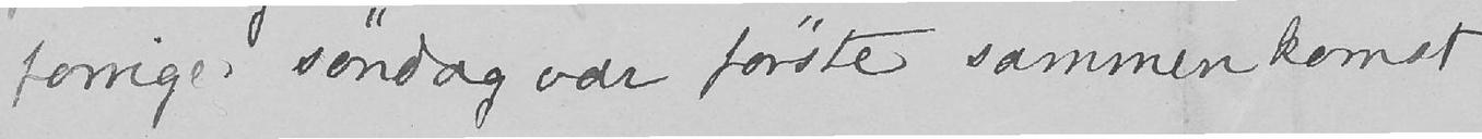forrige søndag var første sammenkomst
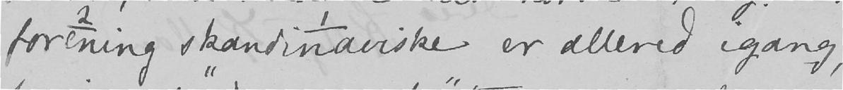forening skandinaviske er allered igang,
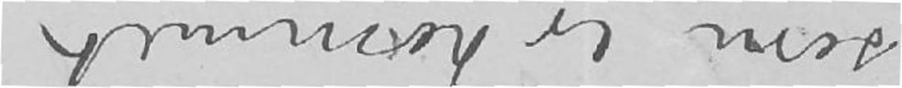som er kommet.
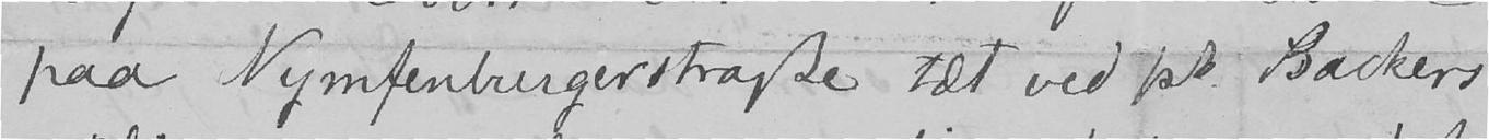paa Nymfenburgerstrasse tæt ved frk. Backers
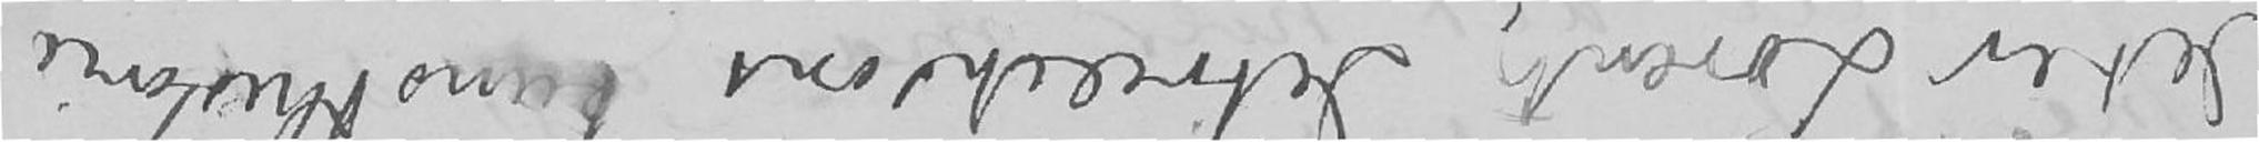Det er Lorentz Ditriechsons kunsthistorie
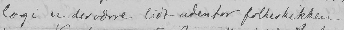logi er desværre lidt udenfor folkeskikken
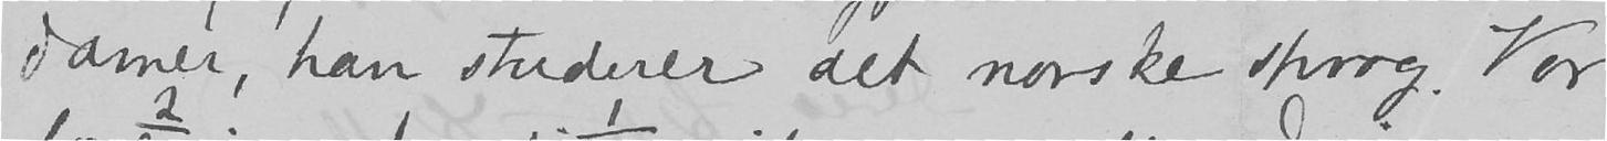damer, han studerer det norske sprog. Vor
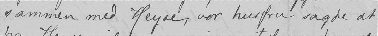sammen med Heyse, vor husfru sagde at
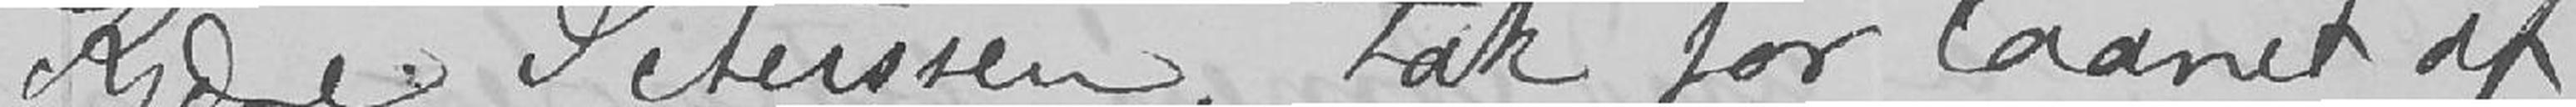Kjære Peterssen, tak for laanet af
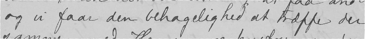og vi faar den behagelighed at træffe der
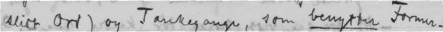slidt Ord) og Tankegange, som benytter Former-
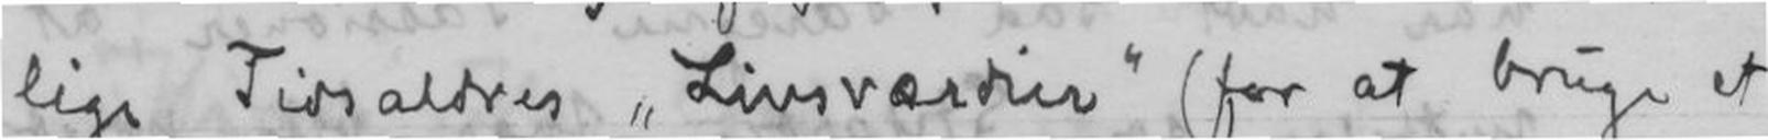lige Tidsaldres Livsværdier (for at bruge et
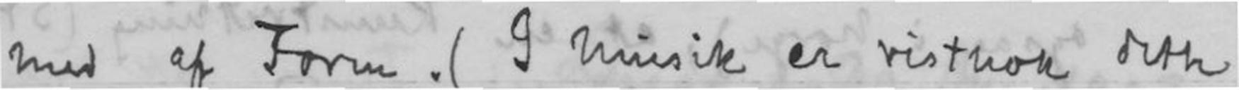med af Form. (I musik er vistnok dette
